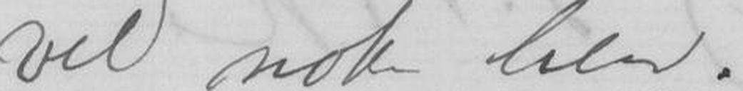vel nok hen.
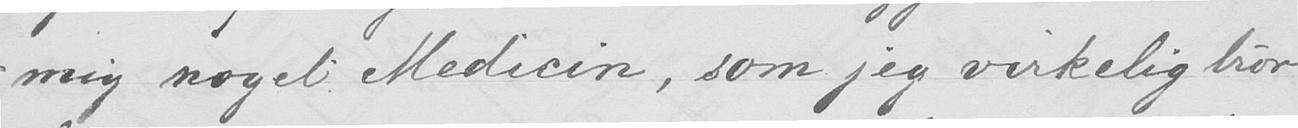mig noget Medicin, som jeg virkelig tror
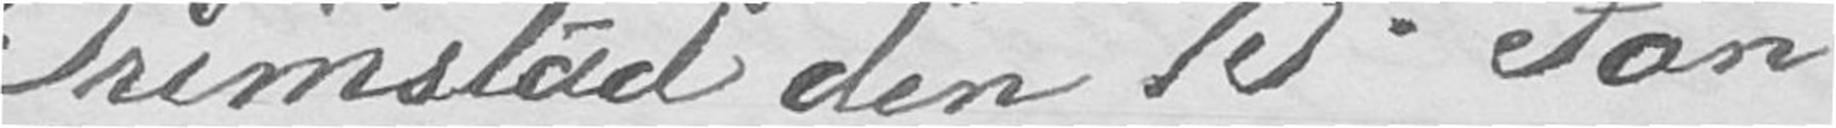Grimstad den 13de Jan
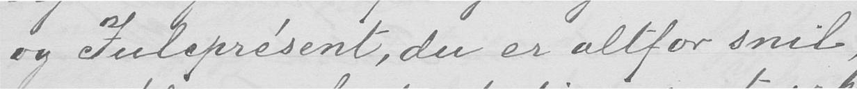og Juleprésent, du er altfor snil,
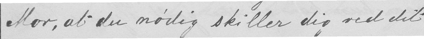Mor, at du nødig skiller dig ved dit
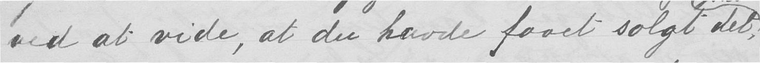ved at vide, at du havde faaet solgt det,
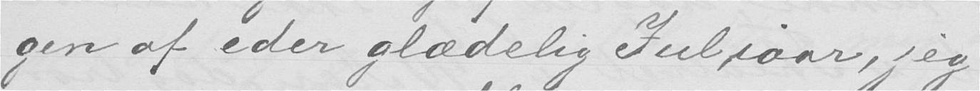gen af eder glædelig Jul iaar, jeg
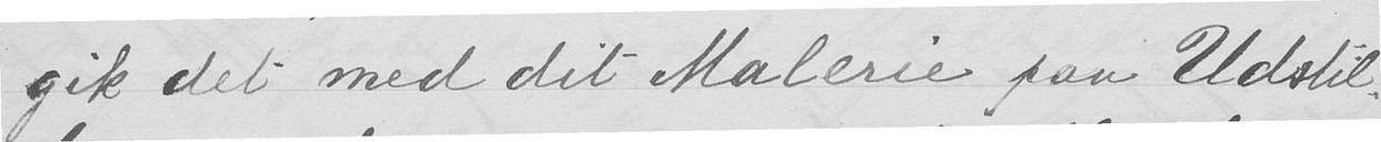gik det med dit Malerie paa Udstil-
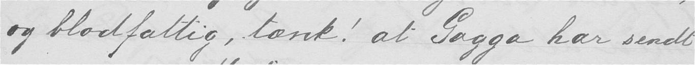og blodfattig, tænk! at Gagga har sendt
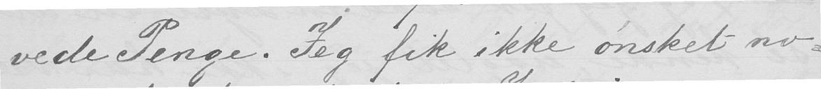vede Penge. Jeg fik ikke ønsket no-
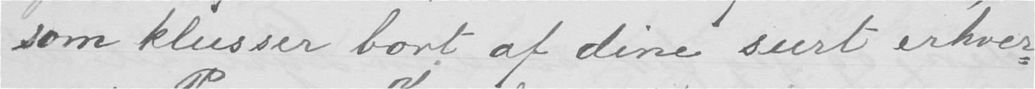som klusser bort af dine surt erhver-
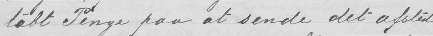tabt Penge paa at sende det afsted
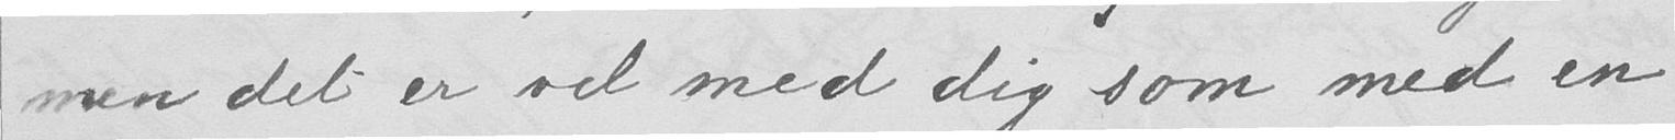men det er vel med dig som med en
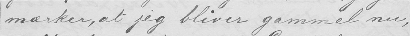mærker, at jeg bliver gammel nu,
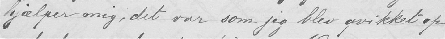hjælper mig, det var som jeg blev qvikket op
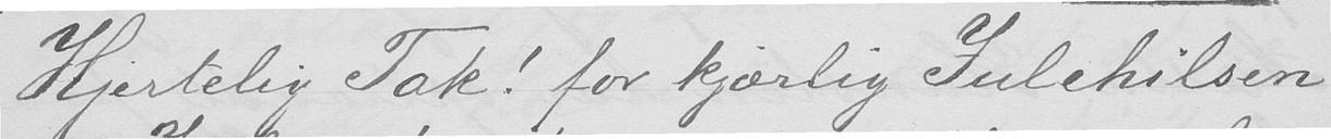Hjertelig Tak! for kjærlig Julehilsen
